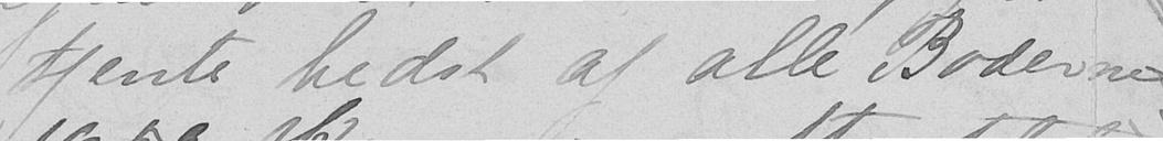tjente bedst af alle Boderne
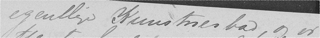egentlige Kunstnerbad, og vi
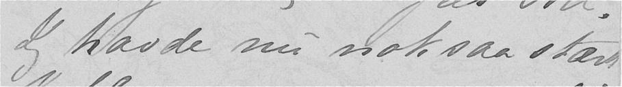Jeg havde nu noksaa stærk
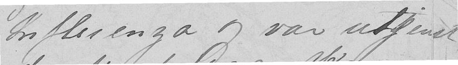Influenza og var utjenst-
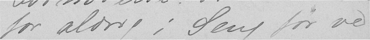for aldrig Seng før ved
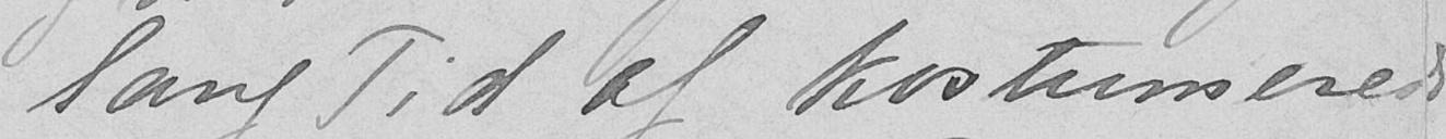lang Tid af kostumerede
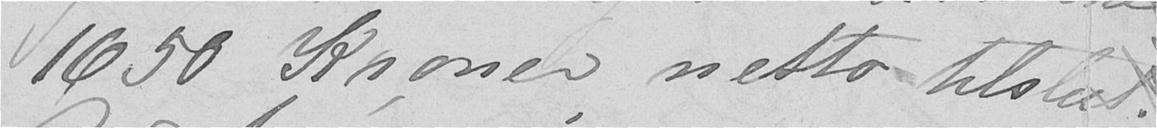1650 Kroner netto tilstut.
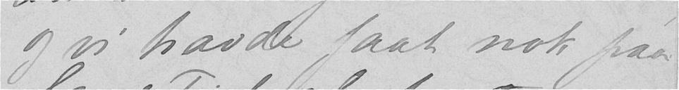og vi havde faat nok paa
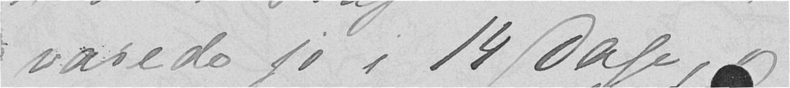varede jo i 14 Dage, og
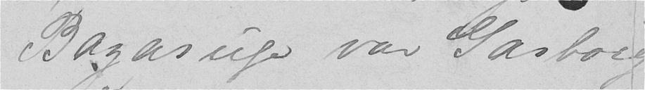Bazaruge var Garborg
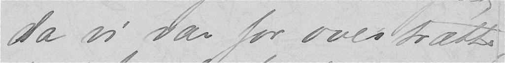da vi var for overtrætte,
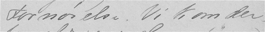Fornøielse. Vi kom der-
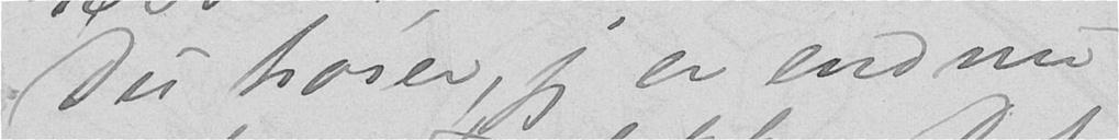Du hører, jeg er endnu
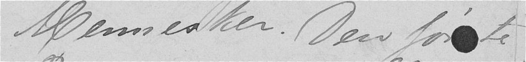Mennesker. Den første
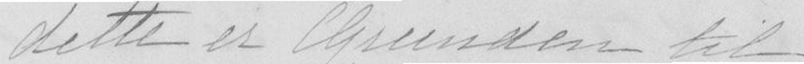dette er Grunden til
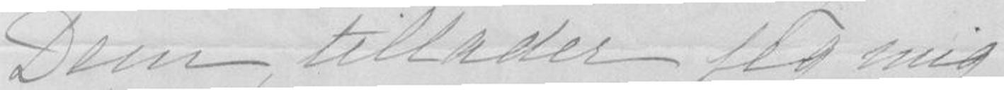Dem, tillader jeg mig
